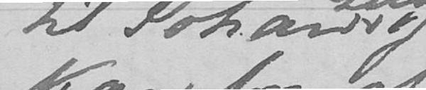til Johan
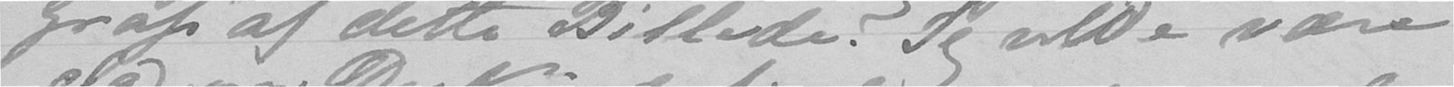grafi af dette Billede? Jeg vilde være
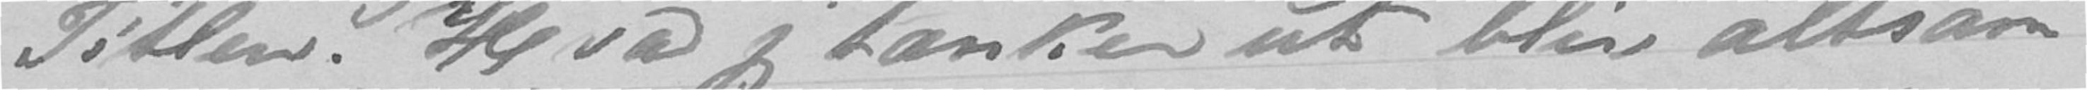Titlen. Hvad jeg tænker ut blir altsam-
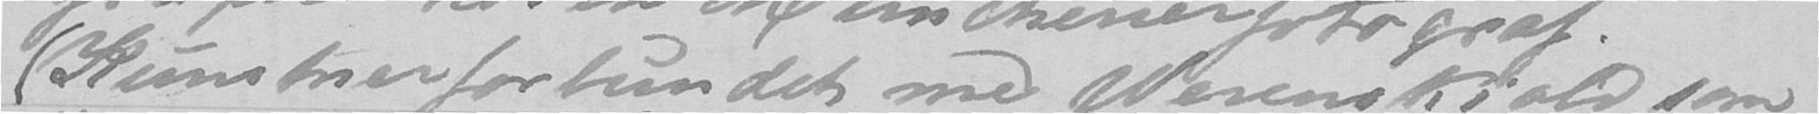(Kunstnerforbundet med Werenskiold som
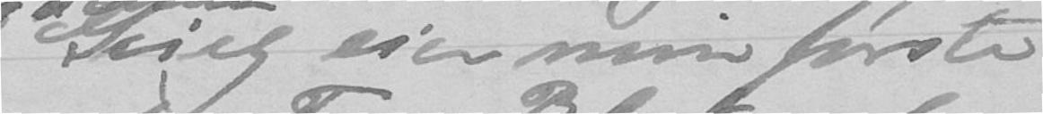Grieg eier min første
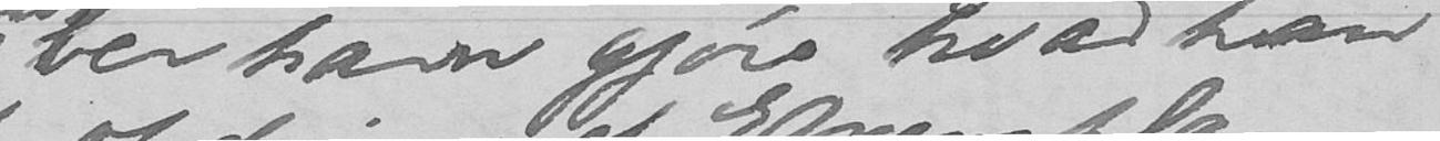og ber ham gjøre hvad han
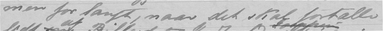men for langt, naar det skal fortælle
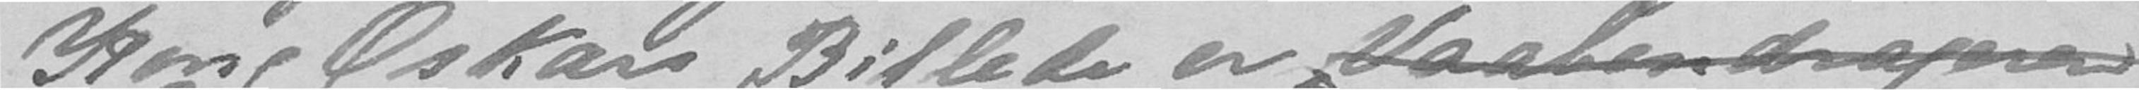Kong Oskars Billede er "Vaabendrageren
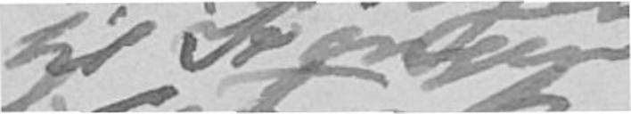til Kongen
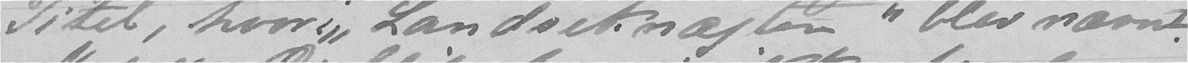Titel, hvori "Landseknægten" blev nævnt.
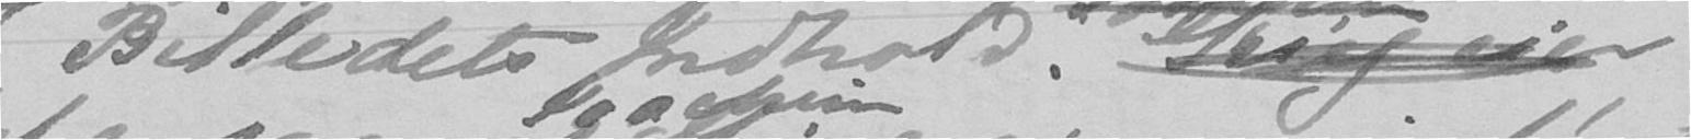Billedets Indhold. Grieg eier
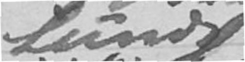Lunde
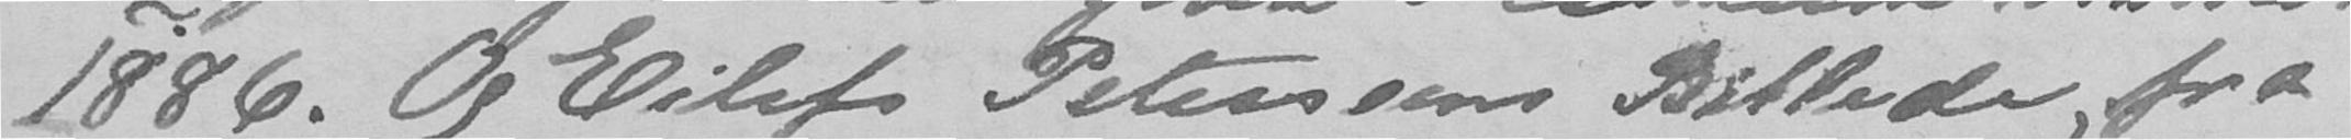1886. Og Eilifs Peterssens Billede, fra
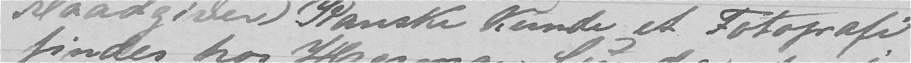Raadgiver) Kanske kunde et Fotografi
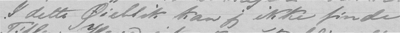I dette Øieblik kan jeg ikke finde
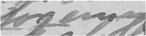forening
